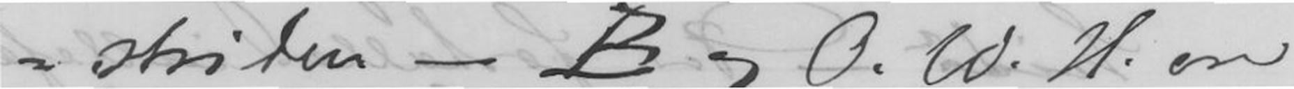striden - B og O. W. H ere
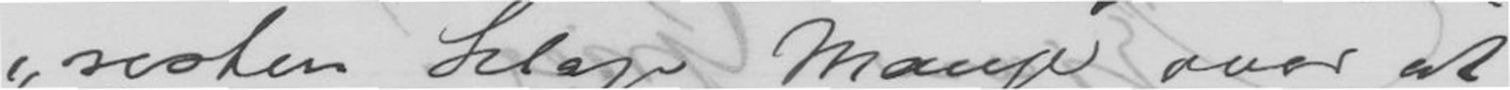resten klager Mange over at
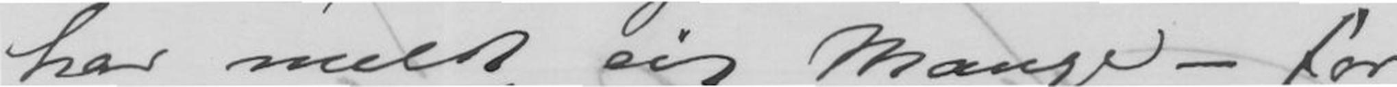har meldt sig Mange - for
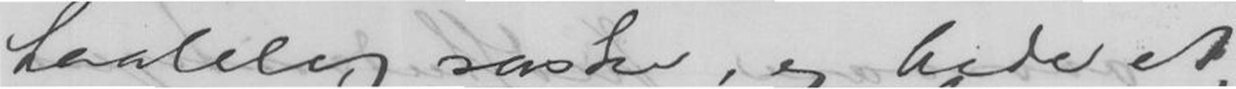taalelig raske, og bede at
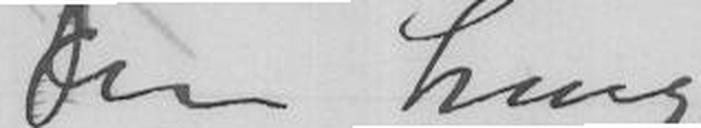Din heng.
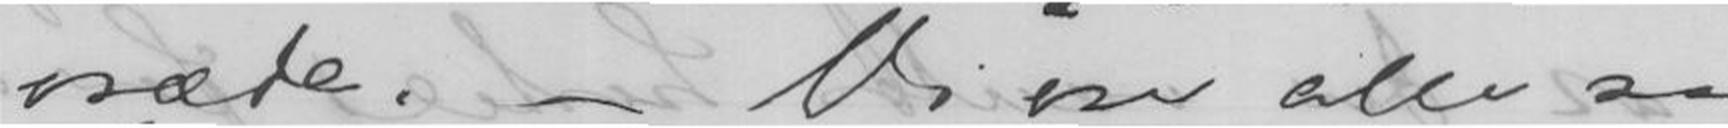vræde. Vi ere alle sa
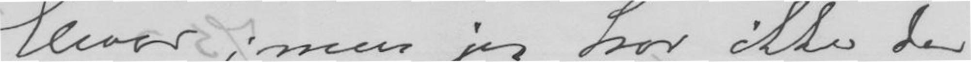Elever; men jeg tror ikke der
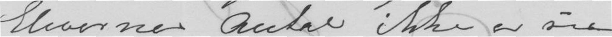Elevernes Antal ikke er saa
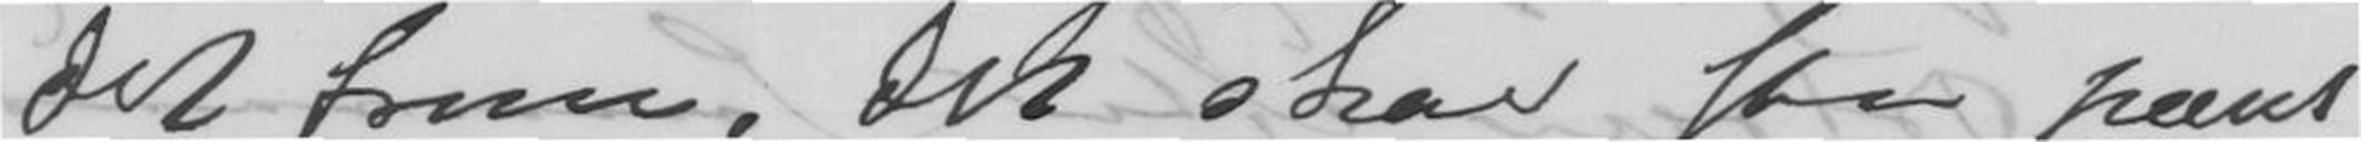det frem. Det skal staa pænt
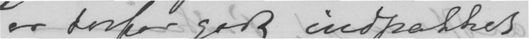er derfor godt indpakket
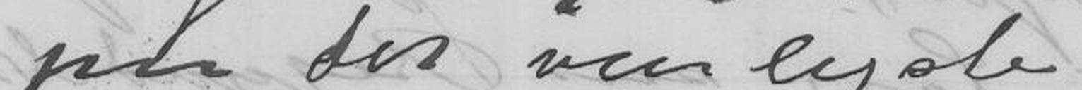paa det venligste
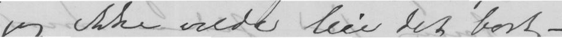jeg ikke vilde leie det bort -
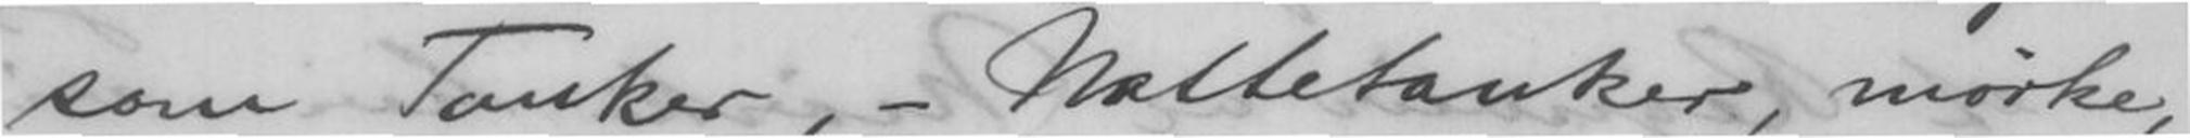som Tanker, - Nattetanker, mørke,
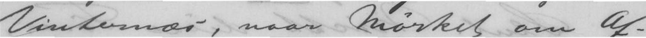Vinternæs, naar Mørket om Af-
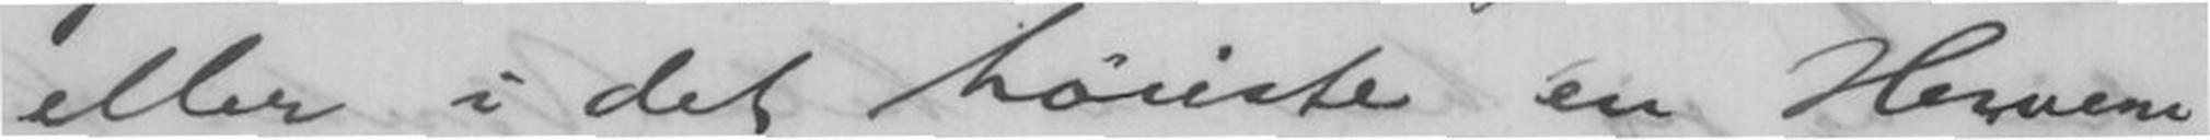eller i det høieste en Henven-
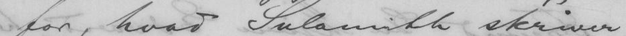for, hvad Sulamith skriver,
